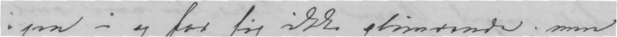gen i og for sig ikke glimrende, men
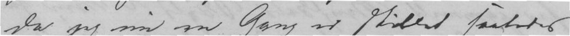da jeg nu en Gang er stillet i saaledes,
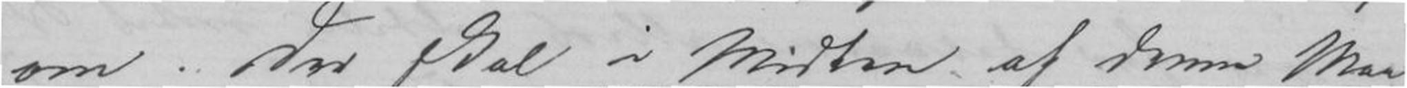om. Du skal i midten af denne maa-
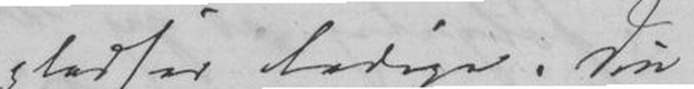pladser ledige.Du
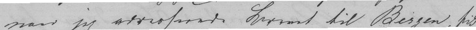naar jeg addresserede Brevet til Bergen, fik
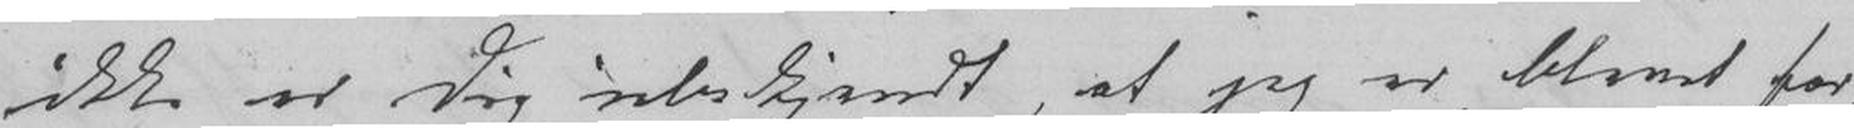ikke er Dig ubekjendt, at jeg er blevet for
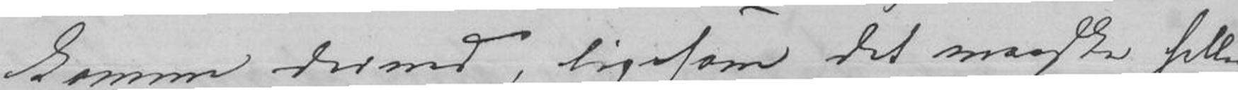komme derned, ligesom det maaske helller
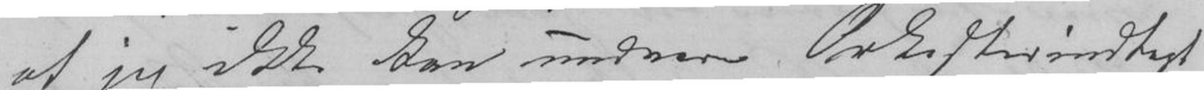at jeg ikke kan undvære Orkesterindtægt,
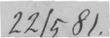22/5 81.
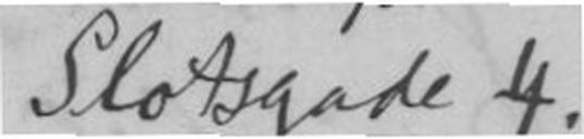Slotsgade 4.
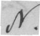N.
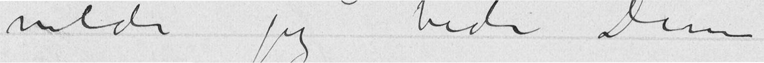vilde jeg bede Dem
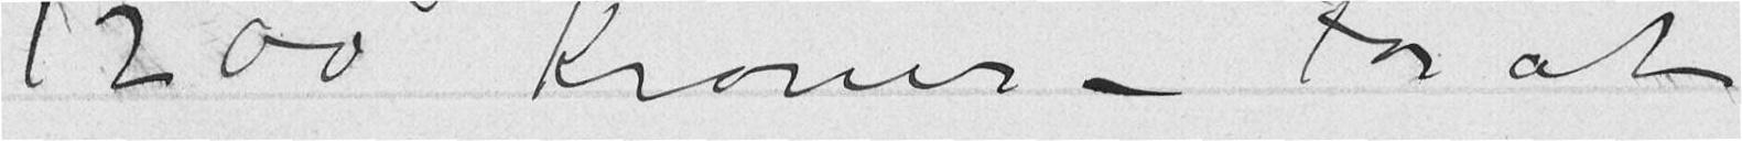1200 Kroner – For at
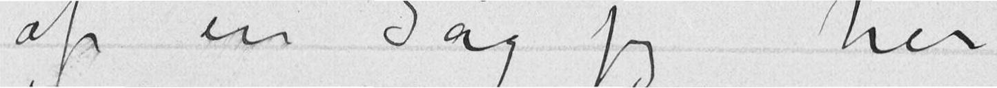af en Sag jeg her
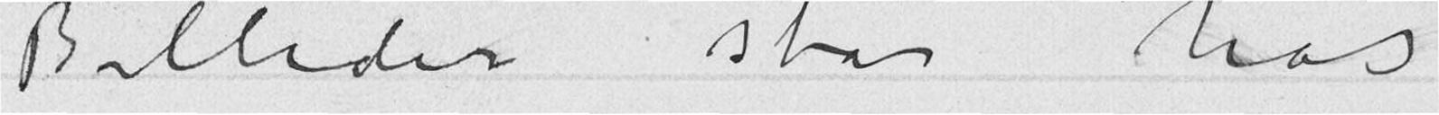Billeder står hos
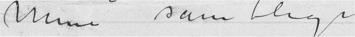Mine samtlige
